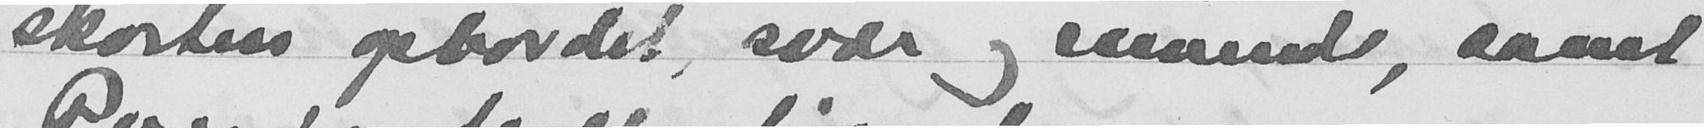skøiten opbordet, svær og ruvende, samt
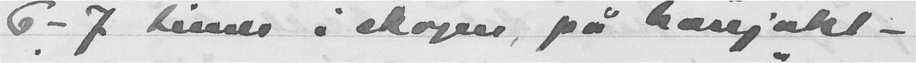6 - 7 timer i skogen, på harejakt -
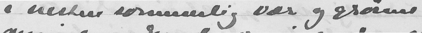i nesten sommerlig vær og grønne
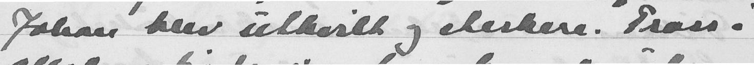Johan blev uthvilt og sterkere. Trass i
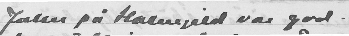Julen på Holmgild var god.
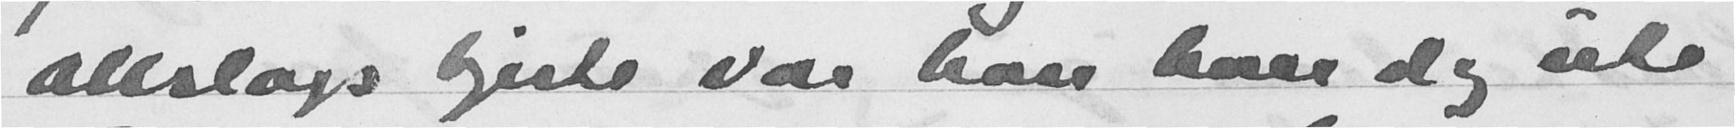allslags hjerte var han hver dag ute
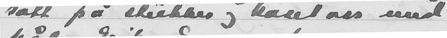satt på skubber og koset oss med
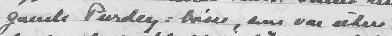gamle Purdey-bøsse, som var uten
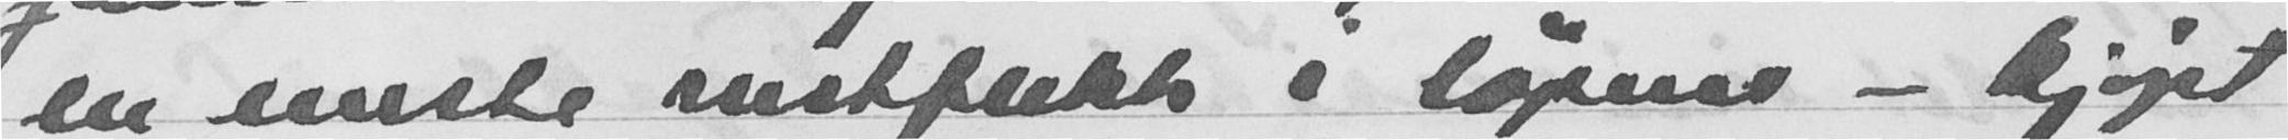en eneste rustflekk i løpene - kjøpt
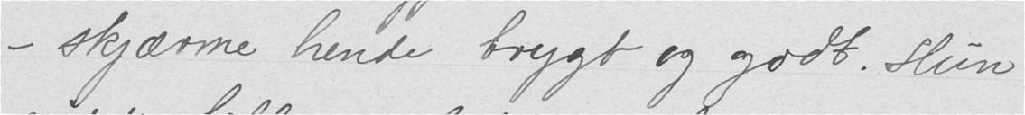– skjærme hende trygt og godt. Hun
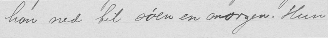hun ned til søen en morgen. Hun
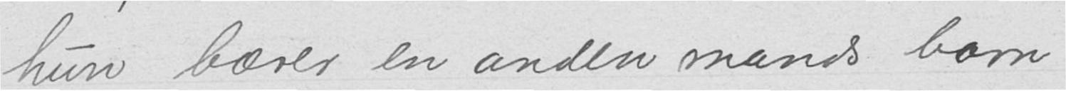hun bærer en anden mands barn.
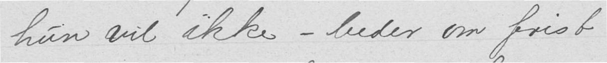hun vil ikke – beder om frist
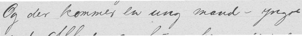Og der kommer en ung mand – yngre
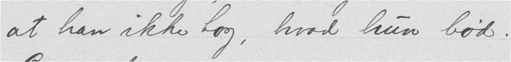at han ikke tog, hvad hun bød.
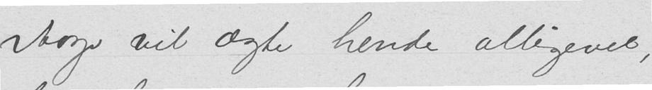Aage vil ægte hende alligevel,
